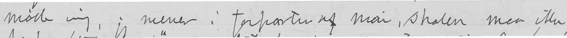møde mig, jeg mener i forparten af mai, skolen maa ikke
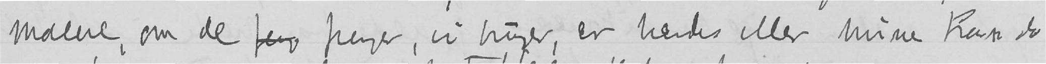malere, om de peg penger, vi bruger, er hendes eller mine kan da
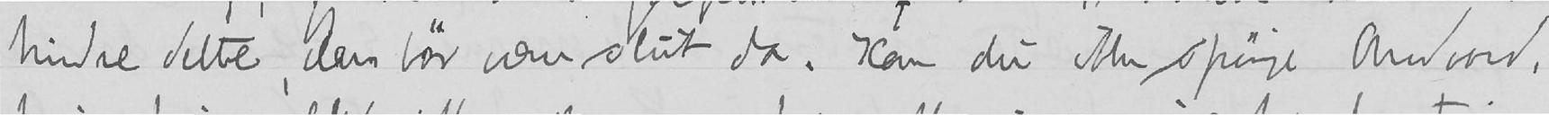hindre dette, den bør være slut da. Kan du ikke spørge Andvord,
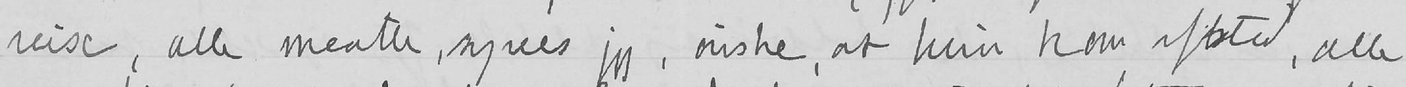reise, alle maatte, synes jeg, ønske, at hun kom afsted, alle
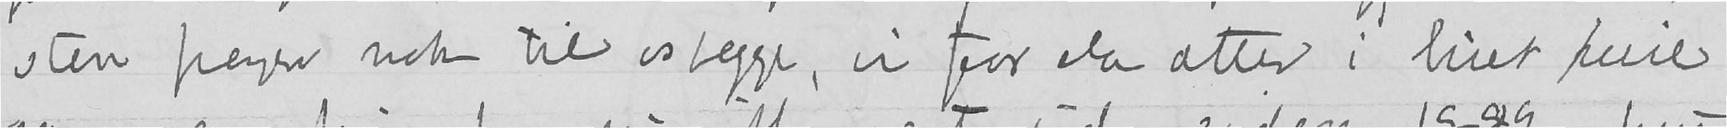sten penger nok til os begge, vi faar da atter i livet ferie
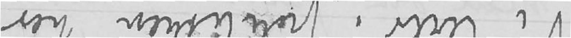Vi lever i politikken her
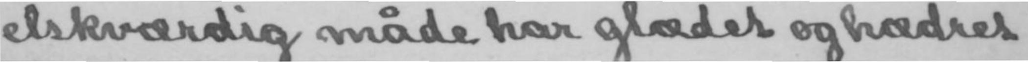elskværdig måde har glædet og hædret
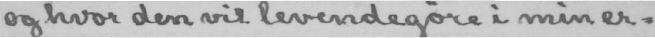og hvor den vil levendegøre i min er-
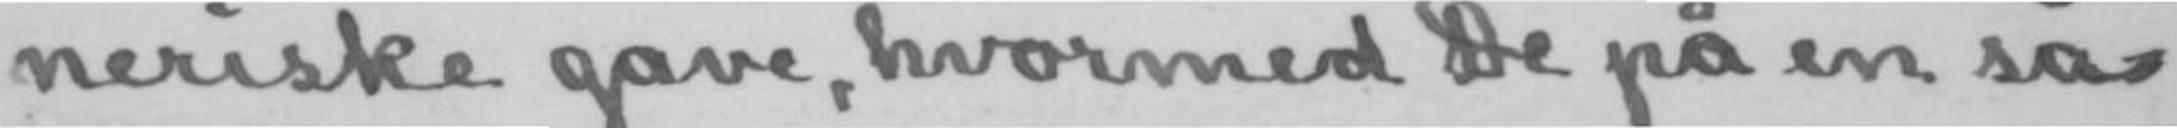neriske gave, hvormed De på en så-
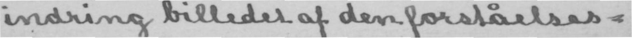indring billedet af den forståelses-
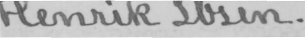Henrik Ibsen.
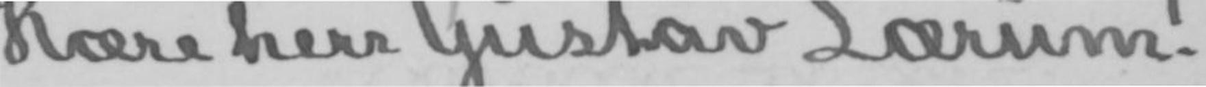Kære herr Gustav Lærum!
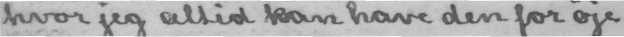hvor jeg altid kan have den for øje
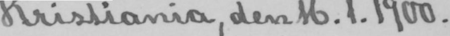Kristiania, den 16.1.1900.
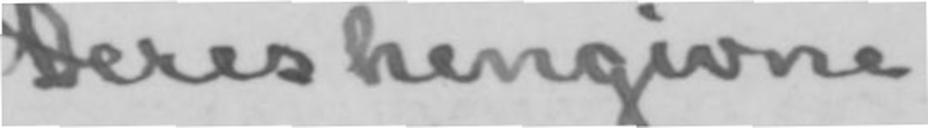Deres hengivne
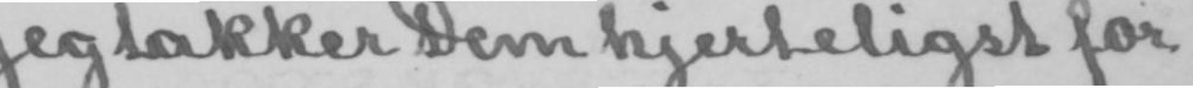Jeg takker Dem hjerteligst for
